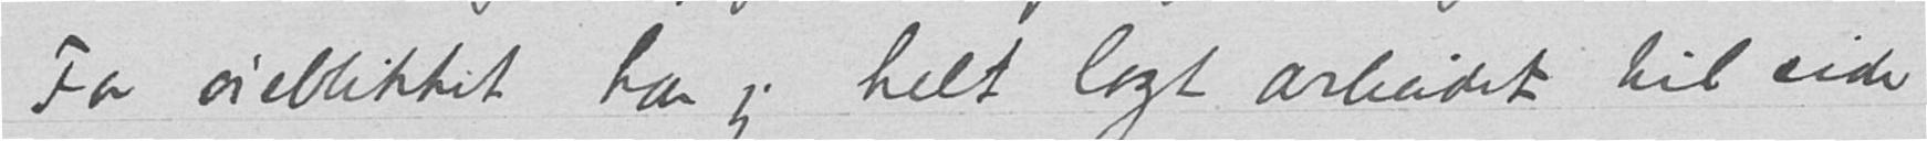For øieblikket har jeg helt lagt arbeidet til side
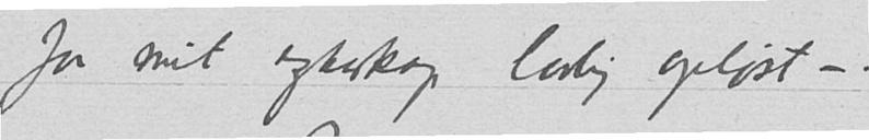faa mit egteskap lovlig opløst –.
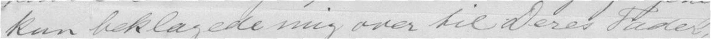kun beklagede mig over til Deres Fader,
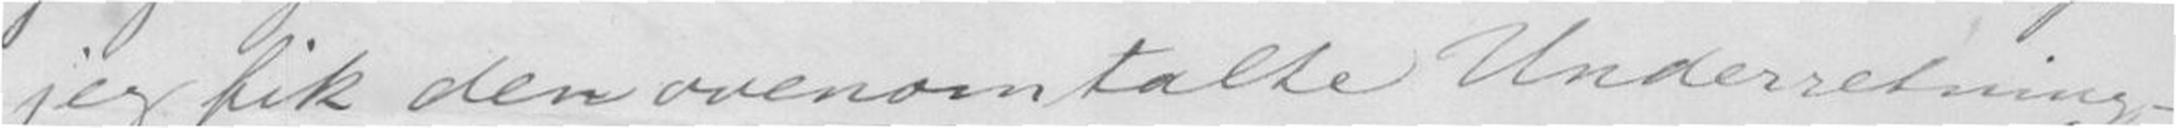før jeg fik den ovenomtalte Underretning -
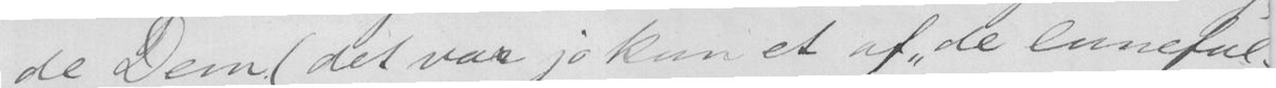de Dem (det var jo kun et af de luneful-
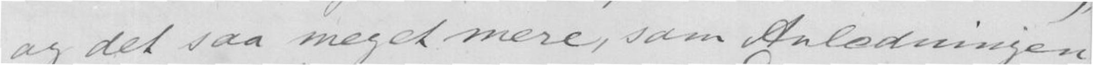og det saa meget mere, som Anlædningen
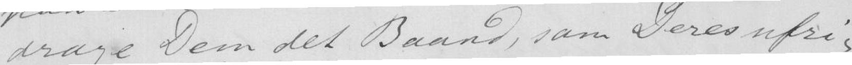drage Dem det Baand, som Deres ufri-
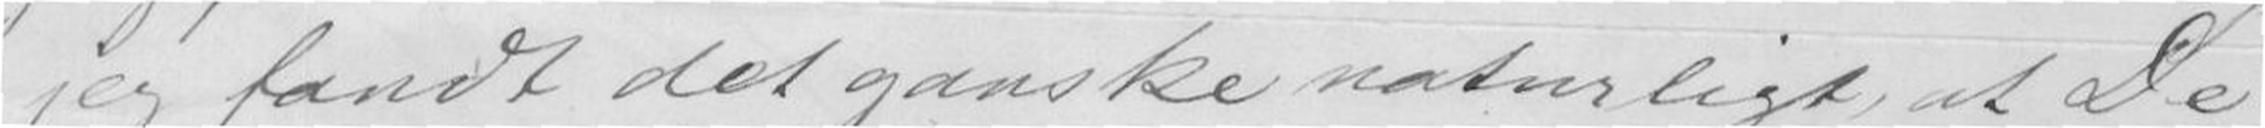jeg fandt det ganske naturlig at De
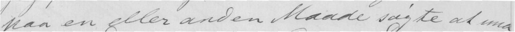paa en eller anden Maade søgte at med-
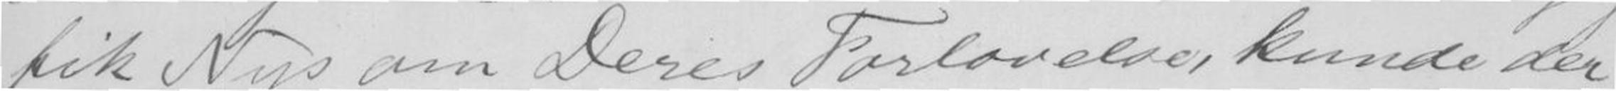fik Nys om Deres Forlovelse, kunde der
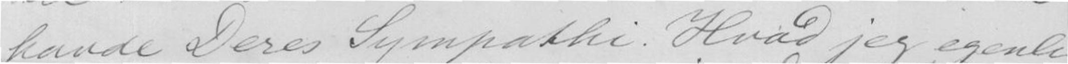havde Deres Sympathi. Hvad jeg egenlig
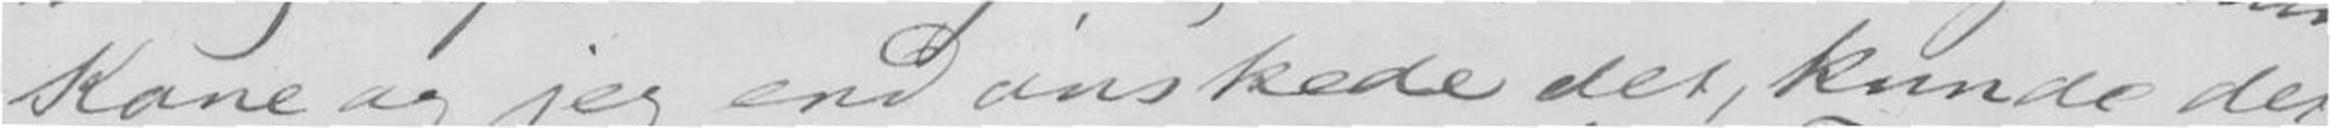Kone og jeg end ønskede det, kunde det
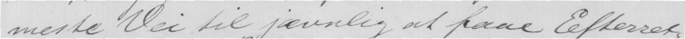meste Vei til jævnlig at faae Efterret-
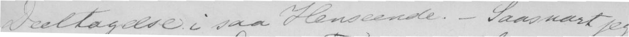Deeltagelse i saa Henseende. - Saasnart jeg
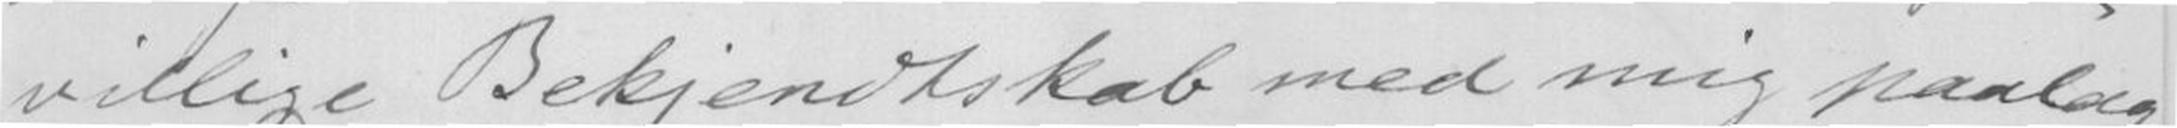villige Bekjendtskab med mig paalag-
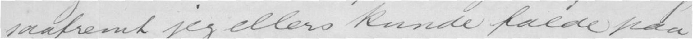saafremt jeg ellers kunde falde paa
